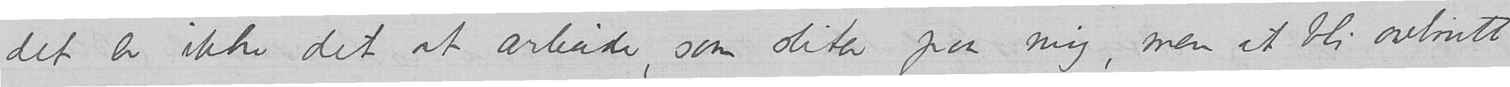det er ikke det at arbeide, som sliter paa mig, men at bli avbrutt
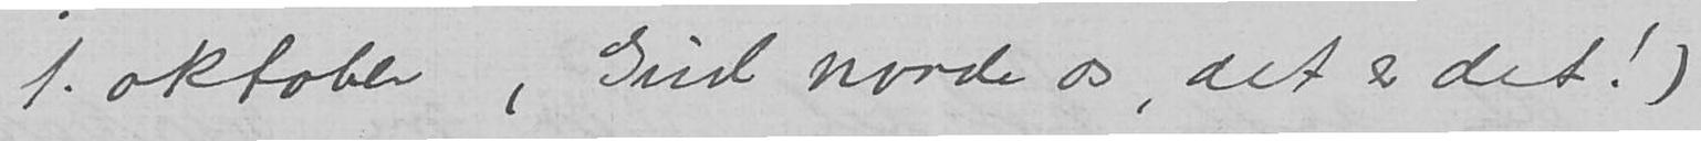1. oktober, Gud naade os, det er det!)
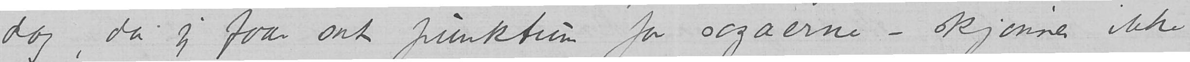dag, da jeg faar sat punktum for sagaerne – skjønner ikke
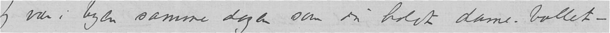Jeg var i byen samme dagen som du holdt dame-ballet –
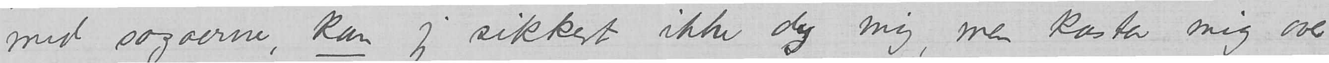med sagaerne, kan jeg sikkert ikke dy mig, men kaster mig over
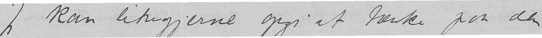Jeg kan likegjerne opgi at tænke paa den
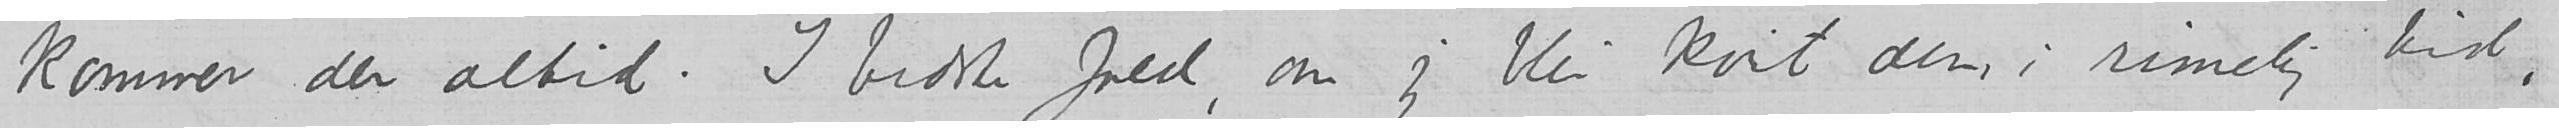kommer der altid. I bedste fald, om jeg blir kvit dem, i rimelig tid,
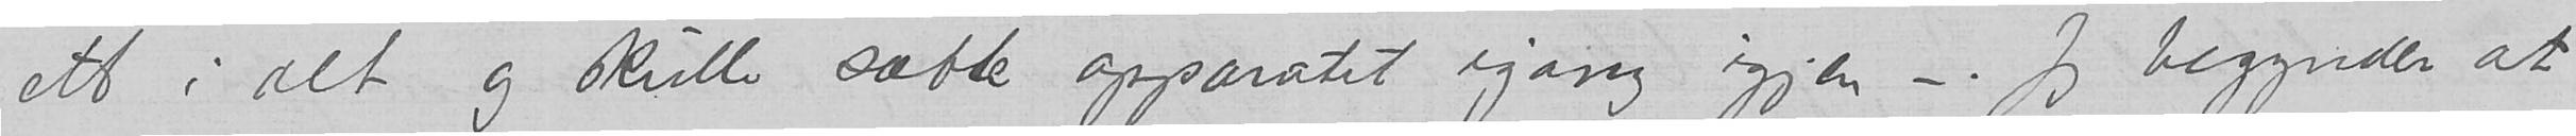ett i alt og skulle sætte apparatet igang igjen –. Jeg begynder at
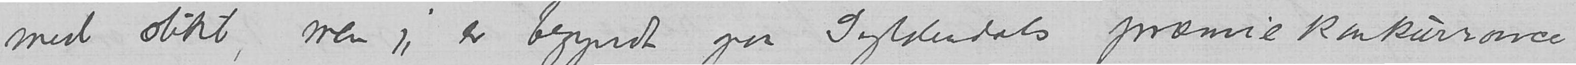med slikt, men jeg er begyndt paa Gyldendals præmiekonkurrance-
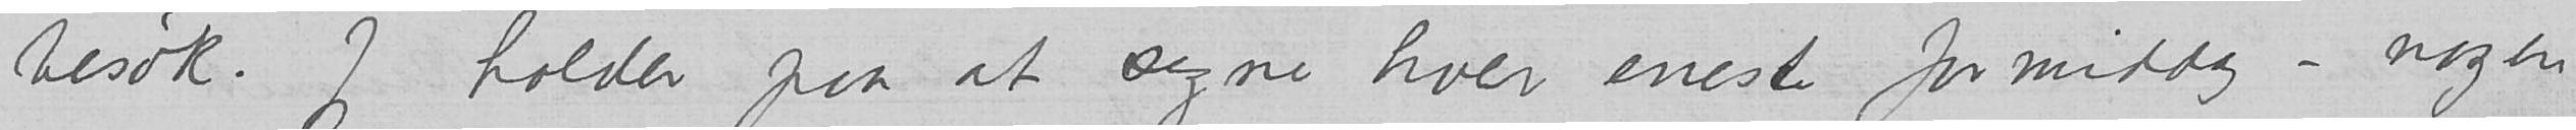besøk. Jeg holder paa at segne hver eneste formiddag – nogen
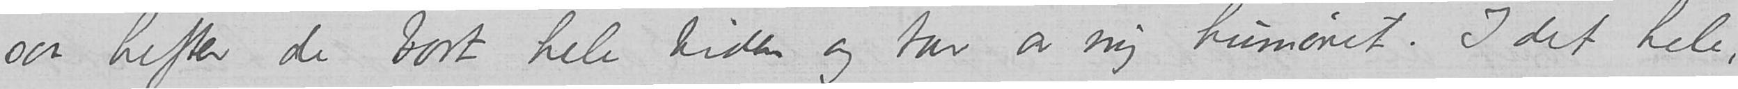saa <hefter> de bort hele tiden og tar av mig humøret. I det hele,
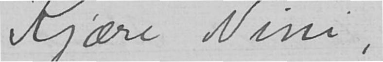Kjære Nini,
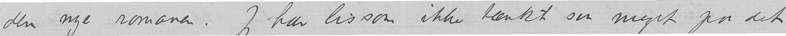den nye romanen. Jeg har lissom ikke tænkt saa meget paa det
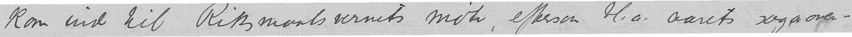kom ind til Riksmaalsvernets møte,  eftersom bl.a. aarets sagaover-
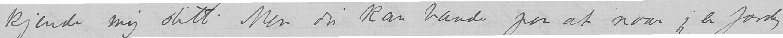kjende mig slitt. Men du kan bande paa at naar jeg er færdig
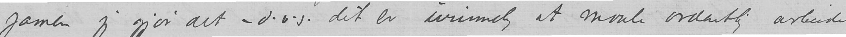jamen jeg gjør det – d.v.s. det er urimelig at maale ordentlig arbeide
